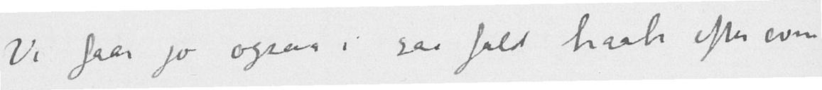Vi faar jo ogsaa i saa fald haabe efter evne
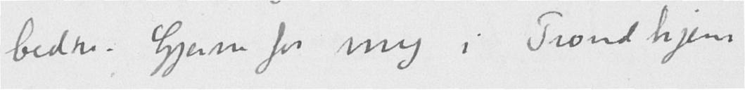bedre. Gjerne for mig i Trondhjem
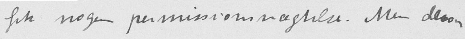fik nogen permissionsnægtelse. Men derom
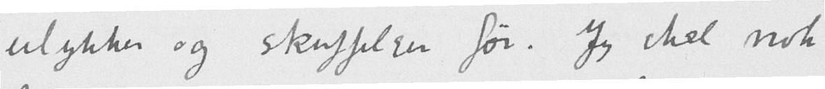ulykker og skuffelser før. Jeg skal nok
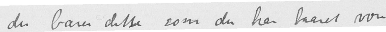du bærer dette som du har baaret vore
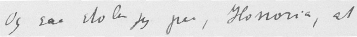Og saa stoler jeg paa, Honoria, at
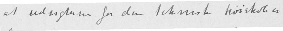at udsigterne for den tekniske høiskole er
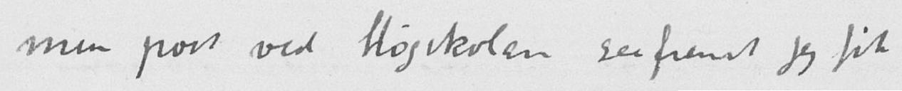min post ved Høgskolan saafremt jeg fik
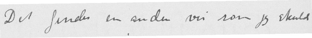Det findes en anden vei som jeg skulde
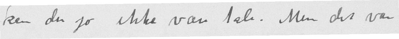kan der jo ikke være tale. Men det var
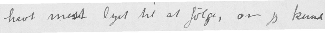havt mest lyst til at følge, om jeg kunde
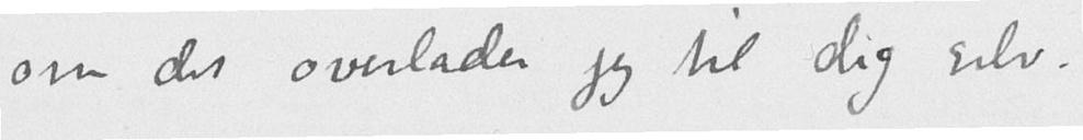om det overlader jeg til dig selv.
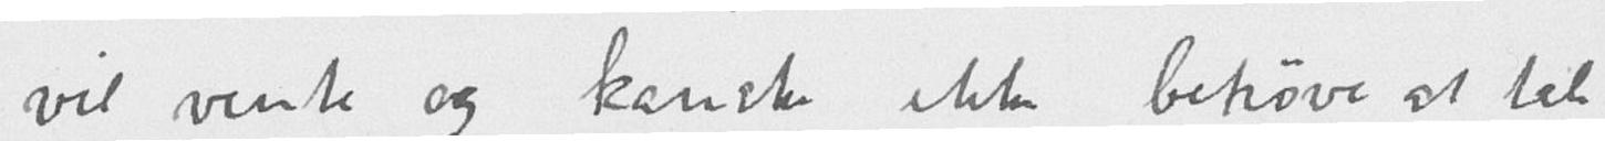vil vente og kanske ikke behøve at tale
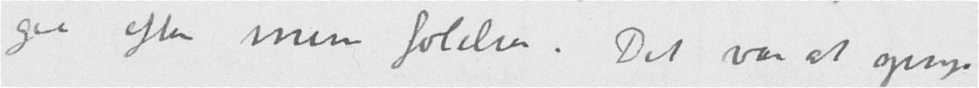gaa efter mine følelser. Det var at opsige
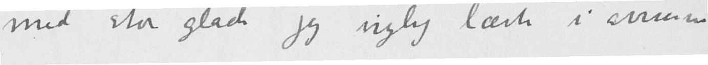med stor glæde jeg nylig læste i aviserne
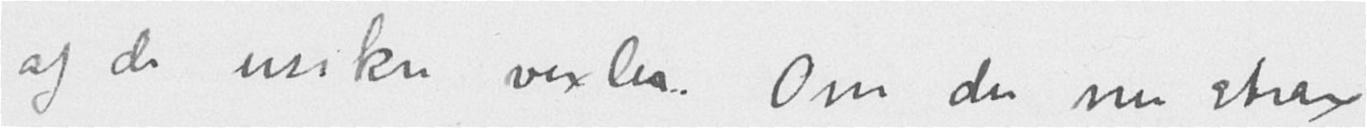af de usikre vexler. Om du nu strax
